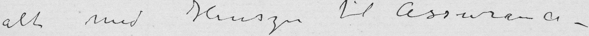alt med Hensyn til Assurance-
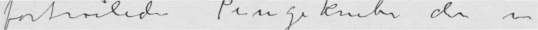fortvivlede Pengeknibe der nu
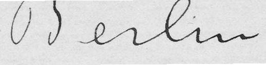Berlin
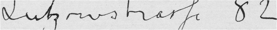Lützowstrasse 82
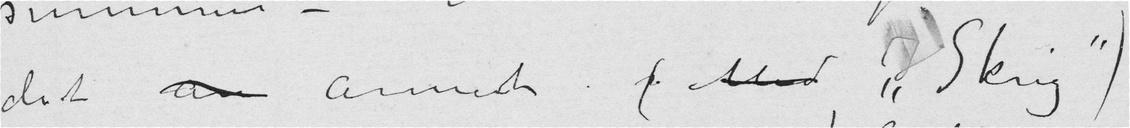det a annet (med H«Skrig»)
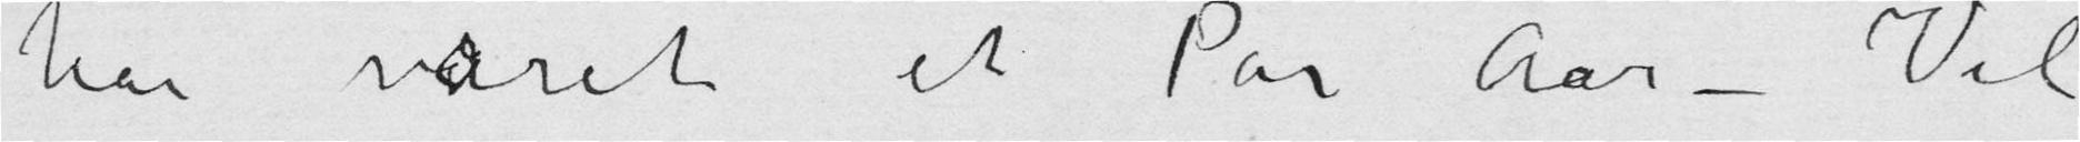har varet et Par Aar – Vil
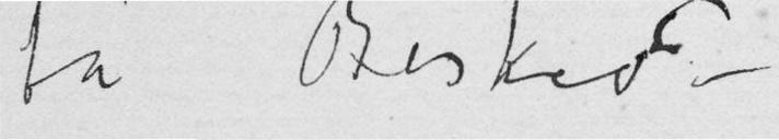få Besked –
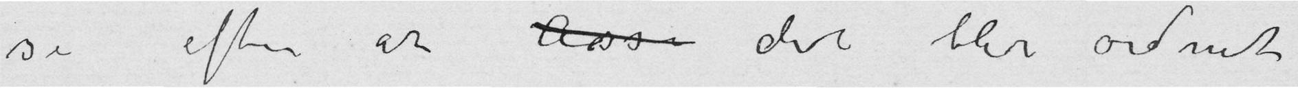se efter at Ass det blir ordnet
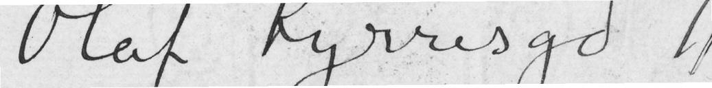Olaf Kyrresgd 11
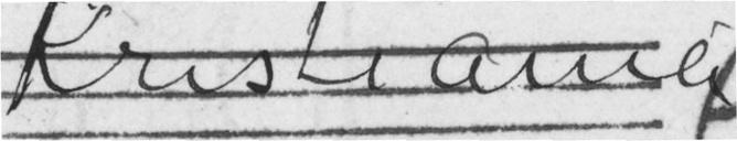Kristiania
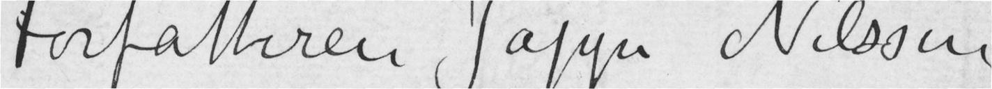Forfatteren Jappe Nilssen
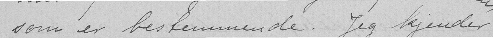som er bestemmende. Jeg kjender
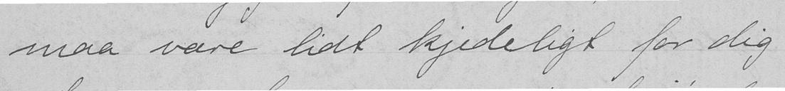maa være lidt kjedeligt for dig
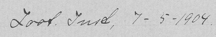Zoot. Inst, 7-5-1904.
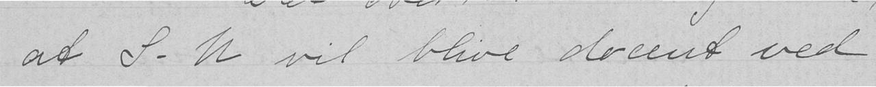at S. N vil blive docent ved
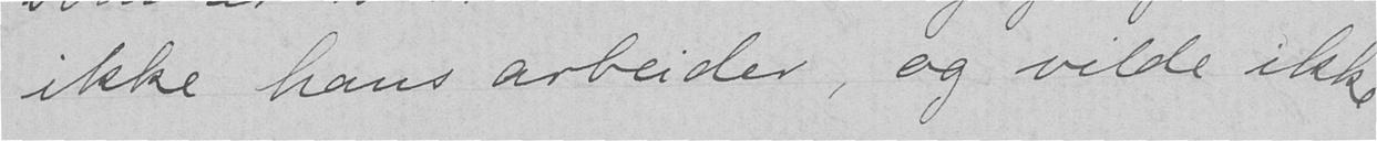ikke hans arbeider, og vilde ikke
